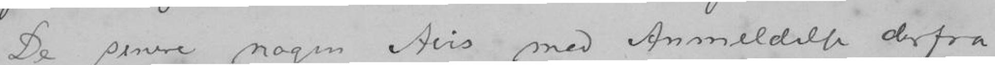De senere nogen Avis med Anmeldelse derfra,
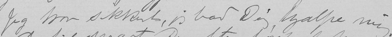Jeg tror sikkert, jeg bad Dig hjælpe mig.
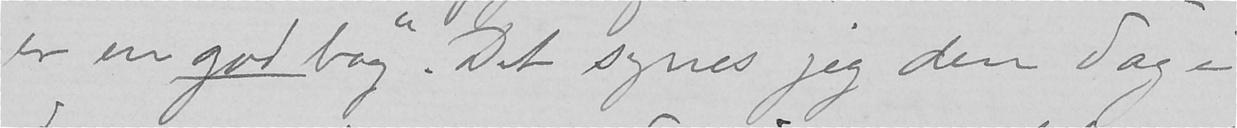er en god bog. Det synes jeg den dag i
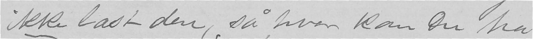ikke læst den, så hvor kan Du ha
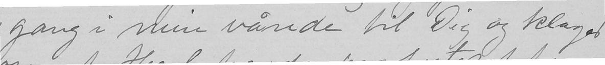gang i min vånde til Dig og klaget
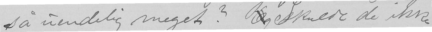så uendelig meget? Og skulde de ikke
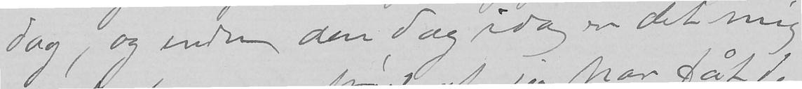dag, og endnu den dag idag er det mig
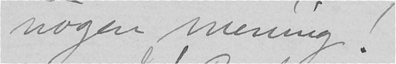nogen mening!
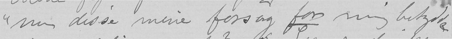nu disse mine forsøg for mig betydde
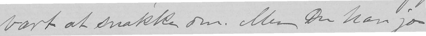vært at snakke om. Men Du har jo
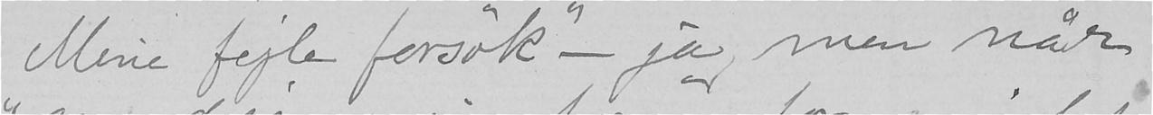"Mine fejle forsøk" - ja, men når
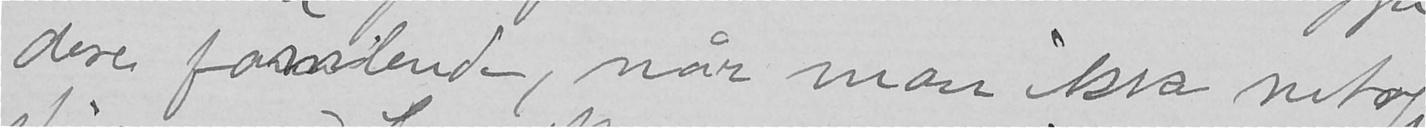dere famlende, når man ikke netop
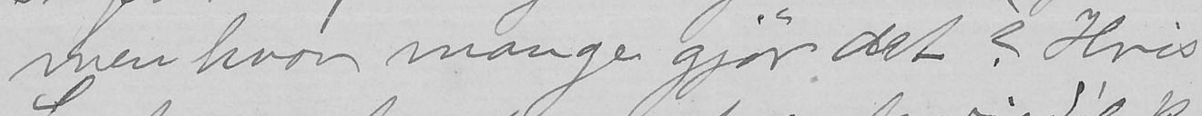men hvor mange gjør det? Hvis
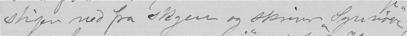stiger ned fra skyen og skriver "Synnøve",
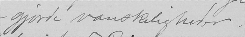gjorde vanskeligheder.
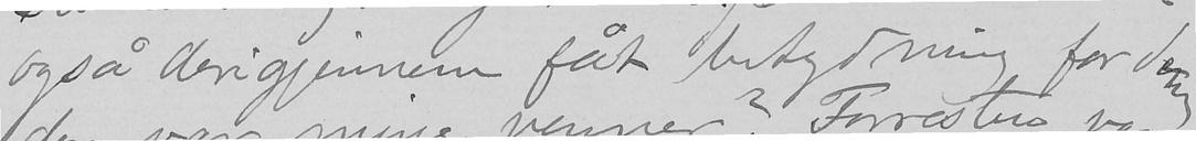også derigjennem fåt betydning for dem
